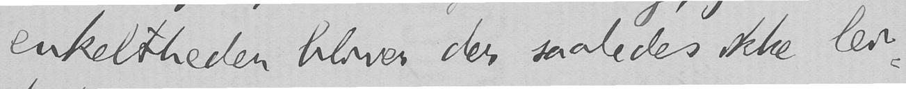enkeltheder bliver der saaledes ikke lei-
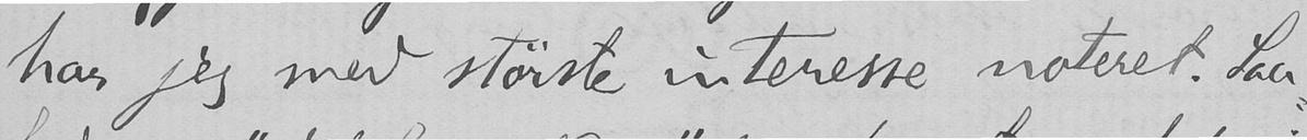har jeg med største interesse noteret. Saa-
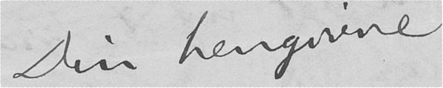Din hengivne
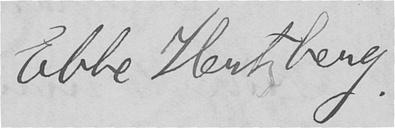Ebbe Hertzberg.
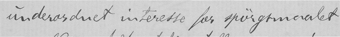underordnet interesse for spørgsmaalet
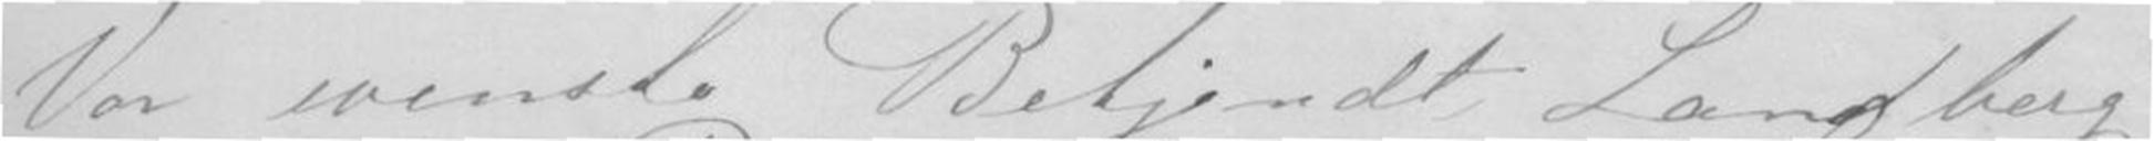Var Bekjendt, Langbeig
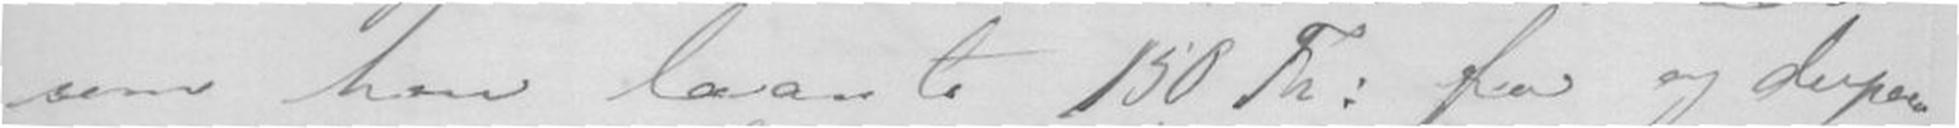som han laante 150 fra og derpaa
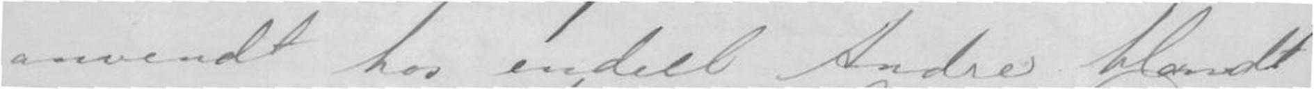anvendt hos endel Andre blandt
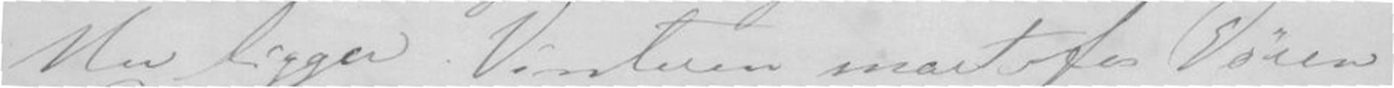Nu ligger Vinteren Døren
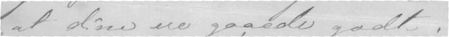at disse ere gaaede godt.
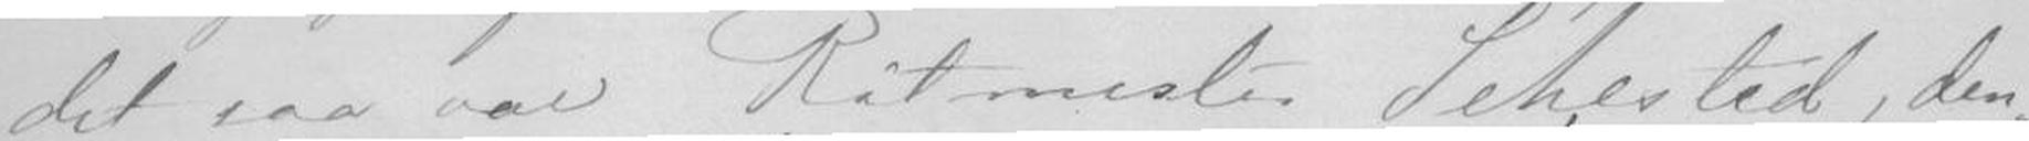det saa var Pitmester Sehested, den-
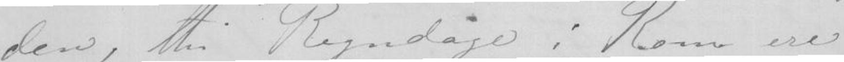den, thi Tegndage i Rom ere
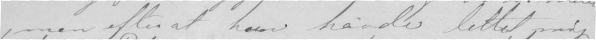,men efter at han havde lettet mig
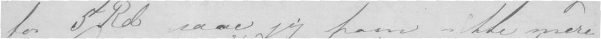for 5 Rd saae jeg ham ikke mere
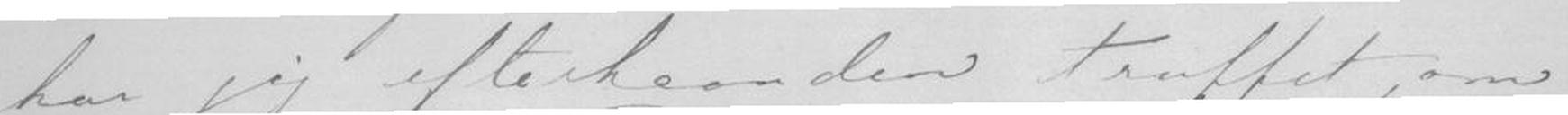har jeg efterhaanden truffet, om
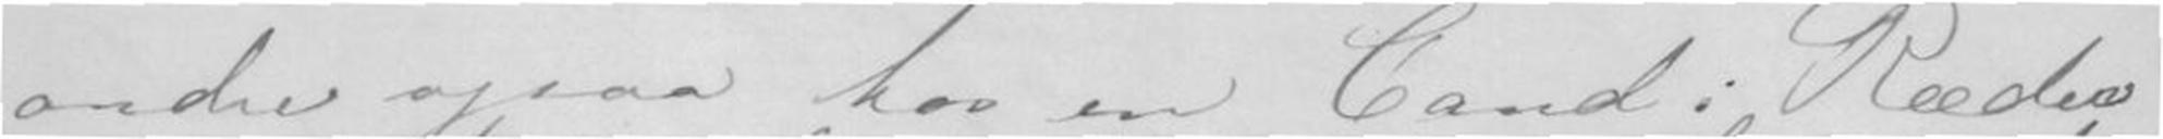andre ogsaa hos en Cand i Ræder
